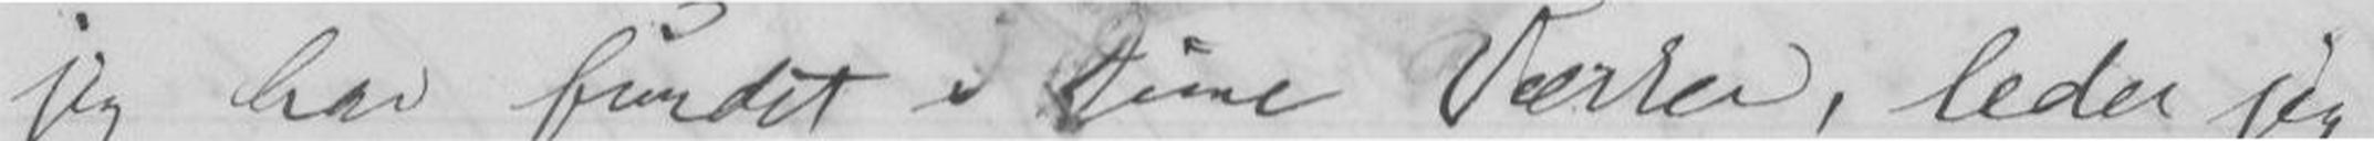jeg har fundet i Dine Værker, leder jeg
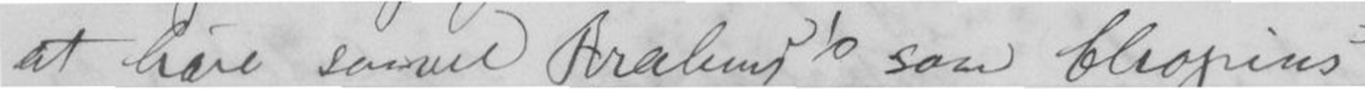at høre samme Brahms's som Chopins
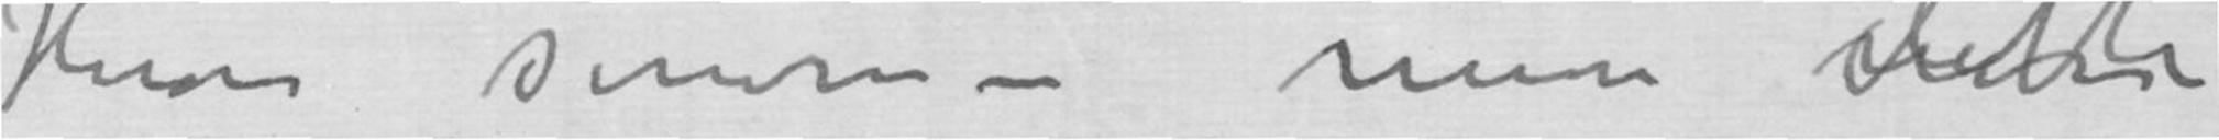Herom senere – men dette
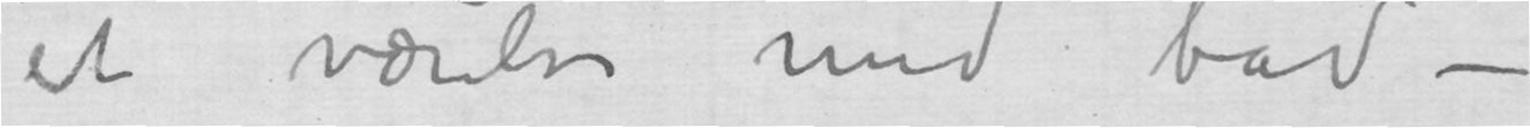et værelse med bad –
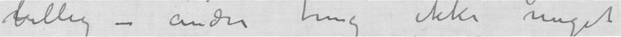billig – andre ting ikke meget
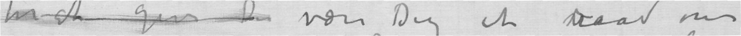for at give D være Dig et raad om
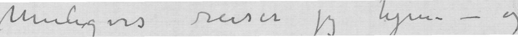Muligens reiser jeg hjem – og
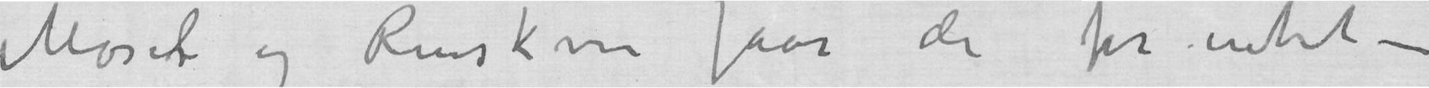Mosel og Rinskvin faar de for intet –
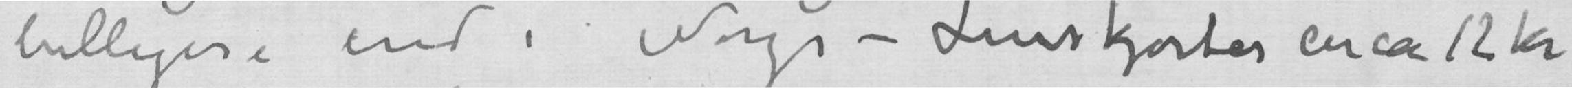billigere end i Norge – Linskjorter en ca 12 Kr
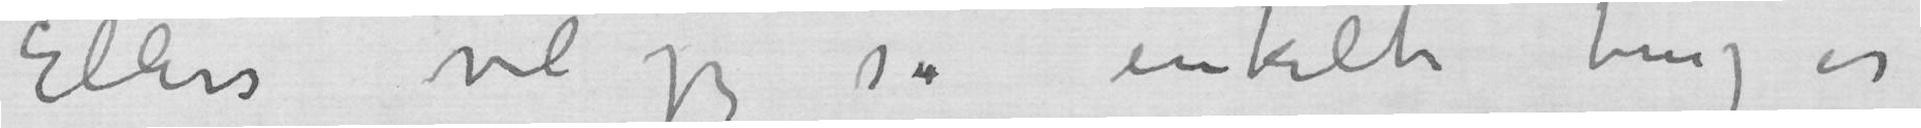Ellers vil jeg si enkelte ting er
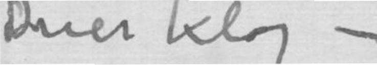Duer klog –
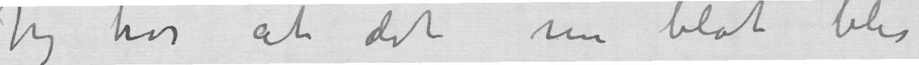Jeg tror at det blot blir
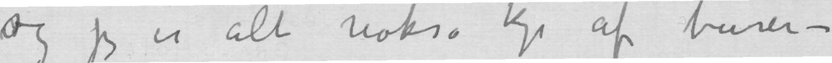og jeg er alt nokså kje af turen –
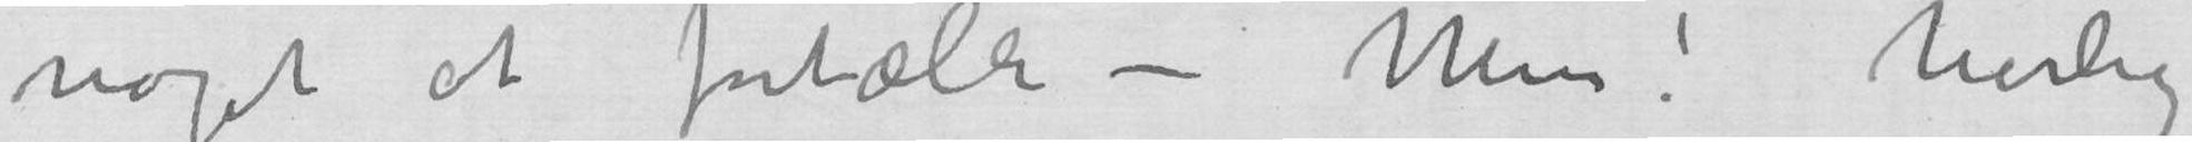noget at fortælle – Men! herlig
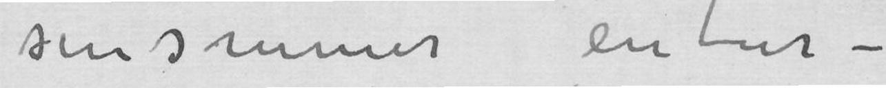sensommer en tur –
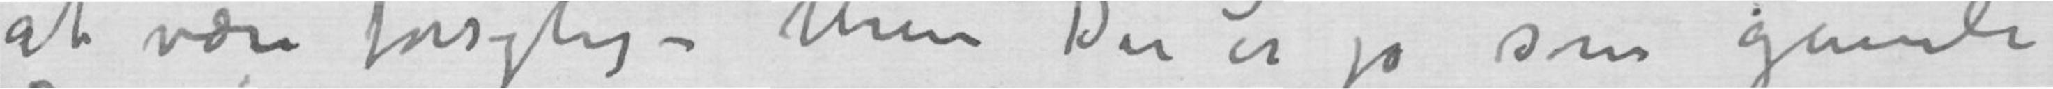at være forsigtig – Men Du er jo som gamle
